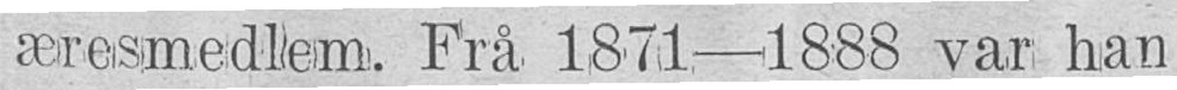æresmedlem. Frå 1871-1888 var han
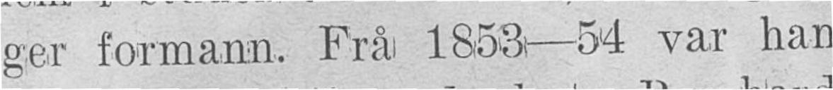ger formann. Frå 1853-54 var han
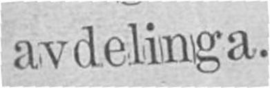avdelinga.
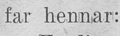far hennar:
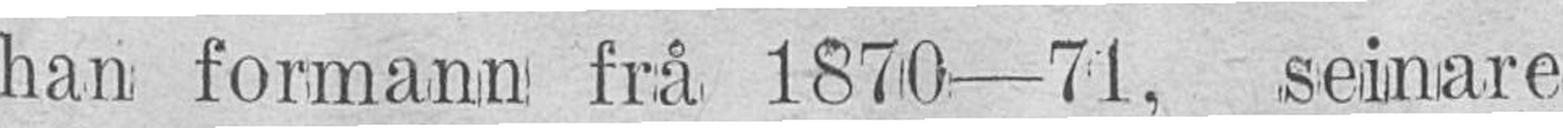han formann frå 1870-71, seinare
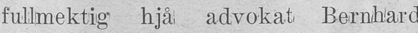fullmektig hjå advokat Bernhard
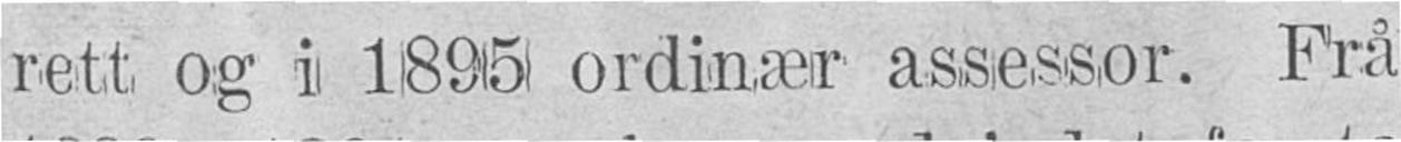rett og i 1895 ordinær assessor. Frå
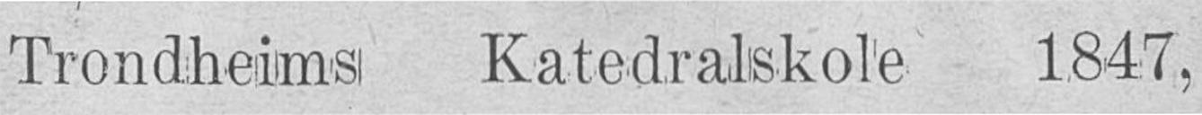Trondheims Katedralskole 1847,
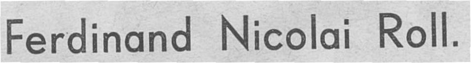Ferdinand Nicolai Roll.
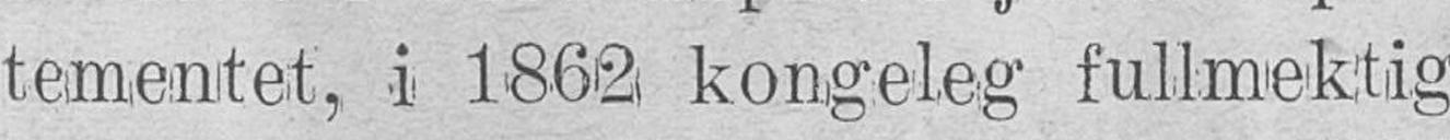tementet, i 1862 kongeleg fullmektig
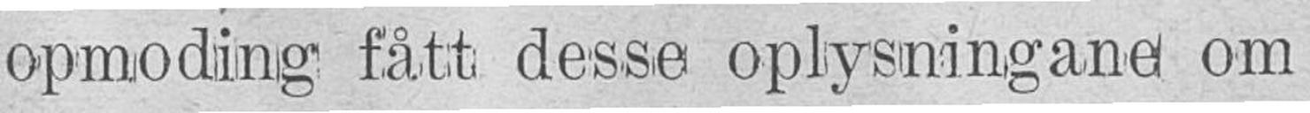opmoding fått desse oplysningane om
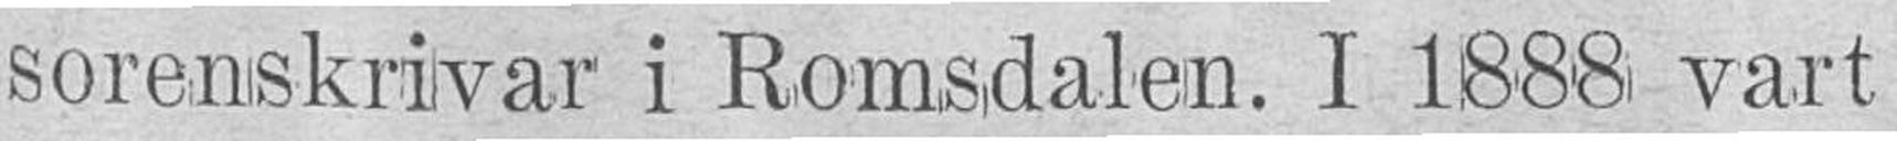sorenskrivar i Romsdalen. I 1888 vart
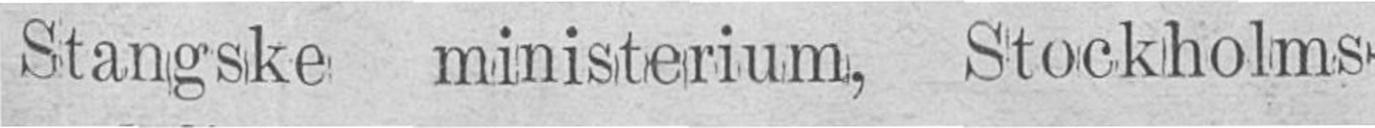Stangske ministerium, Stockholms-
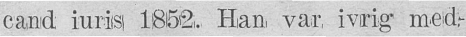cand iuris 1852. Han var ivrig med-
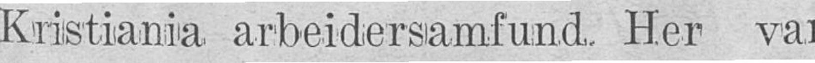Kristiania arbeidersamfund. Her var
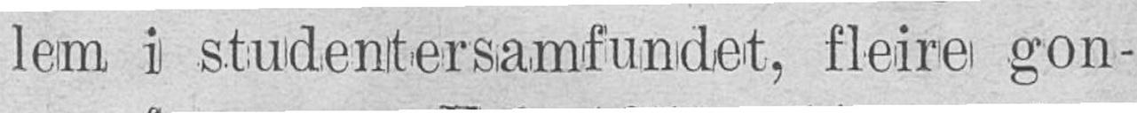lem i studentersamfundet, fleire gon-
